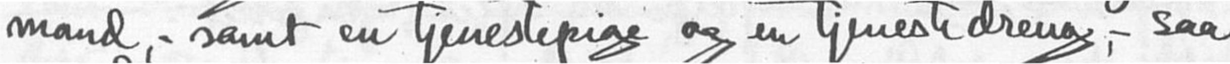mand, - samt en tjenestepige og en tjenestedreng, - saa
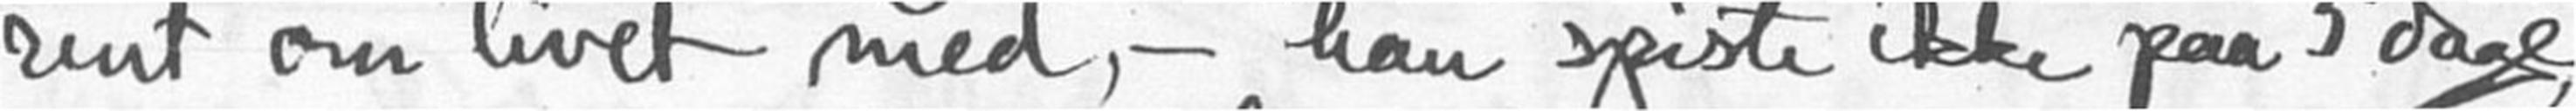rent om livet med, - han spiste ikke paa 5 dage
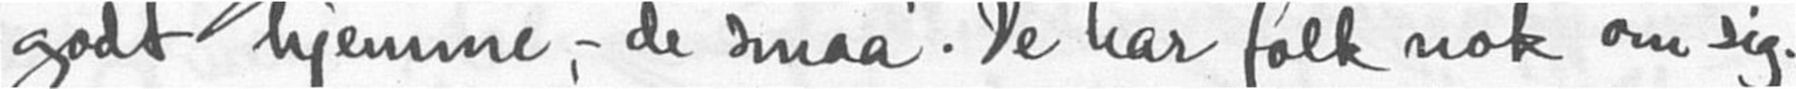godt hjemme, - de smaa. De har folk nok om sig.
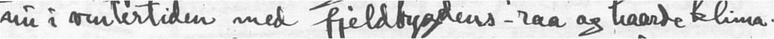nu i vintertiden med fjeldbygdens raa og haarde klima.
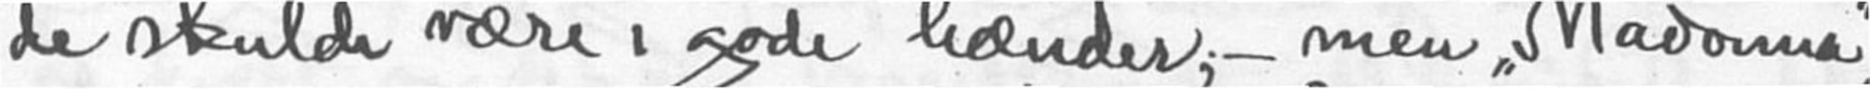de skulde være i gode hænder, - men "Madonna"
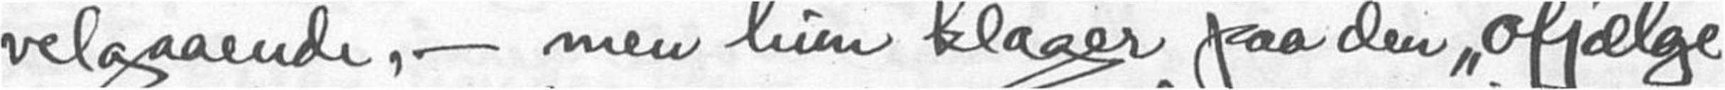velgaaende, - men hun klager paa den "ofjælge
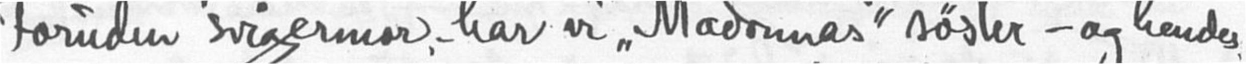Foruden svigermor, har vi "Madonnas" søster - og hendes
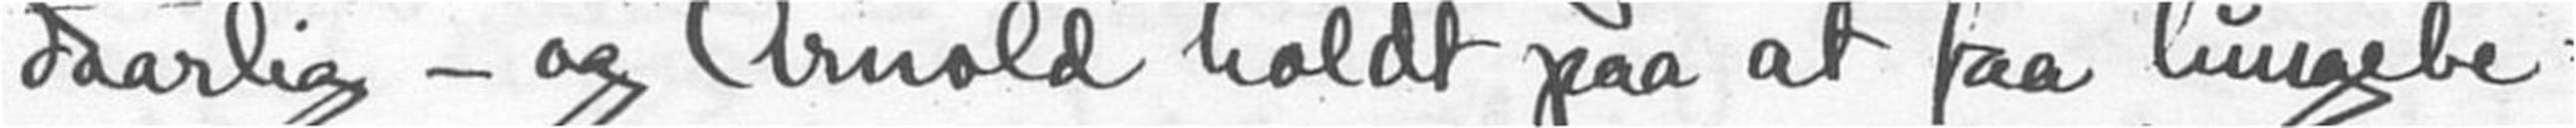daarlig - og Arnold holdt paa at faa lungebe-
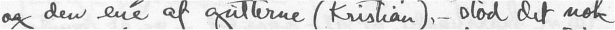og den ene af gutterne (Kristian), - stod det nok
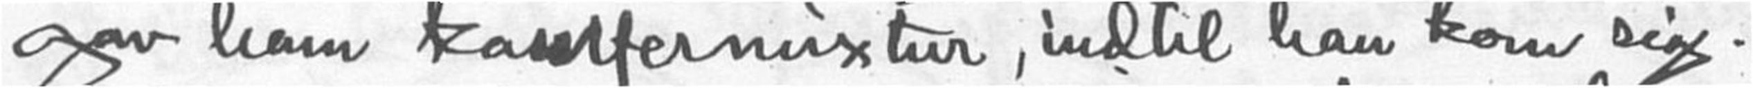gav ham kamfermixtur, indtil han kom sig.
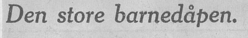Den store barnedåpen.
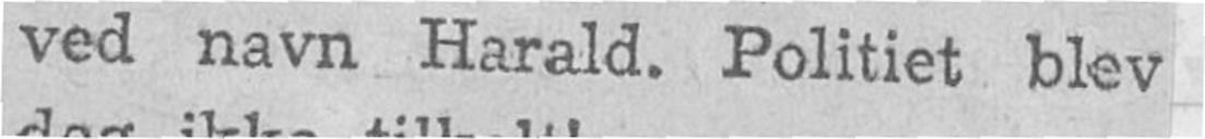ved navn Harald. Politiet blev
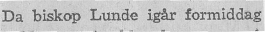Da biskop Lunde igår formiddag
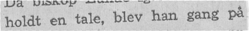holdt en tale, blev han gang på
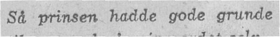Så prinsen hadde gode grunde
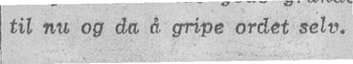til nu og da å gripe ordet selv.
